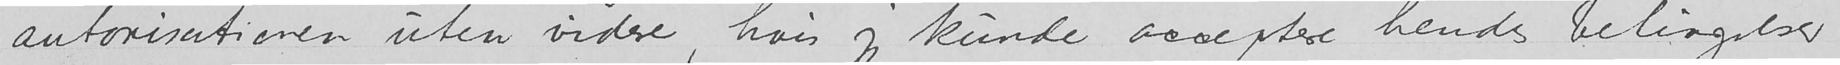autorisationen uten videre, hvis jeg kunde acceptere hendes betingelser.
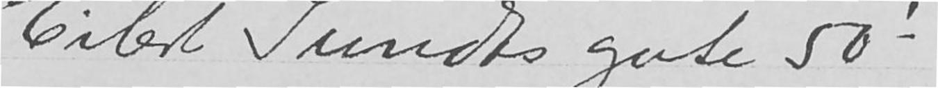Eilert Sundts gate 50
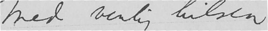Med venlig hilsen
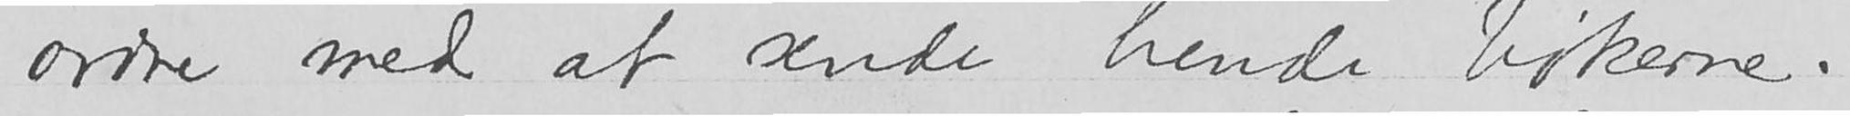ordne med at sende hende bøkerne.
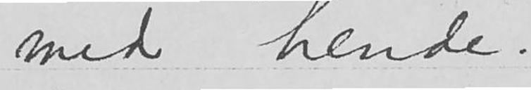med hende.
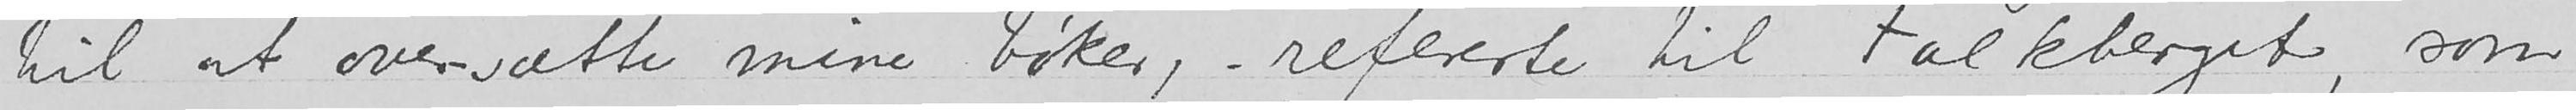til at oversætte mine bøker, - refererte til Falkberget, som
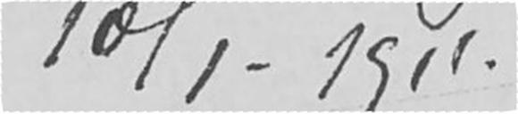10/1-1911
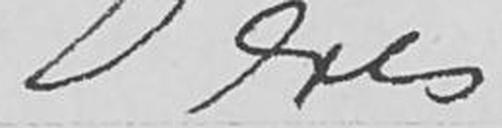Deres
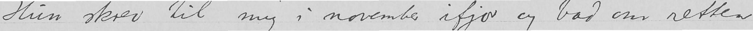Hun skrev til mig i november ifjor og bad om retten
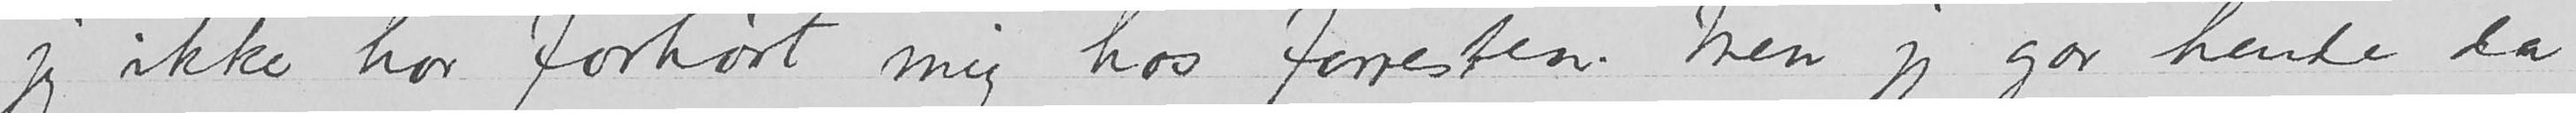jeg ikke har forhørt mig hos forresten. Men jeg gav hende da
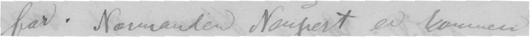for. Normanden Neupert er kommen
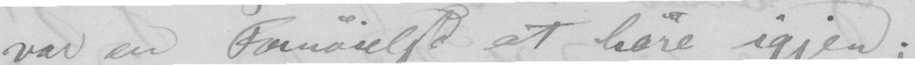var en Fornøielse at høre igjen;
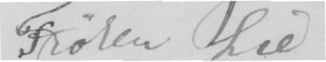Frøken Lie
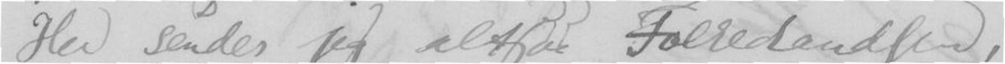Her sender jeg altsaa Folkedandsen,
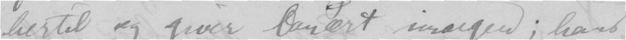hertil og giver Consært imorgen; hans
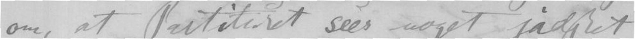om, at Partituret seer noget jadsket
